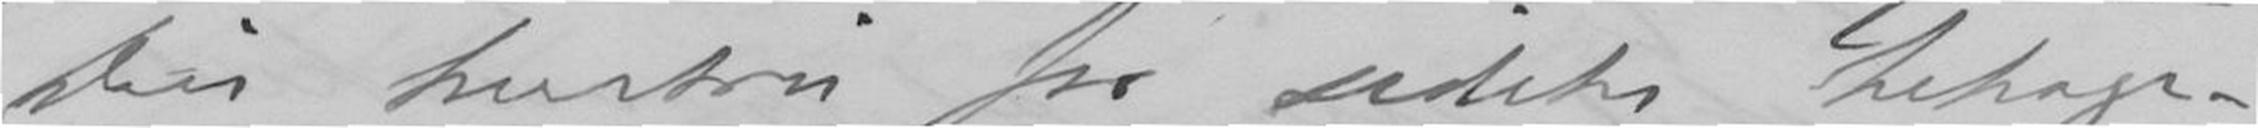Din hustru for sidste behage-
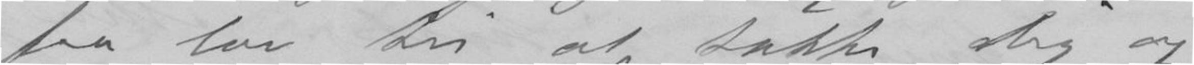faa lov til at takke Dig og
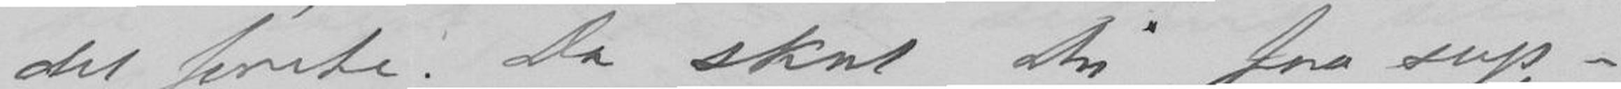det første. Da skal Du faa supp-
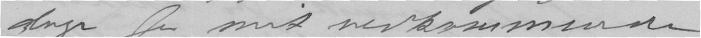dage for mit vedkommende
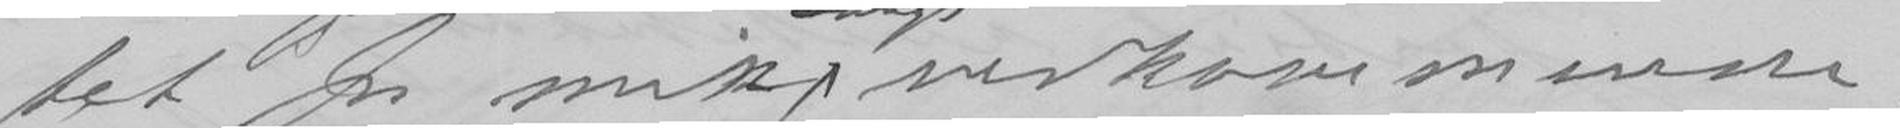tet for min vedkommende.
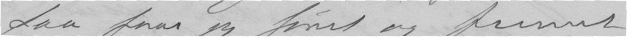Saa faar jeg først og fremst
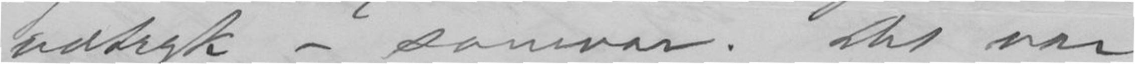udtryk - samvær. Det var
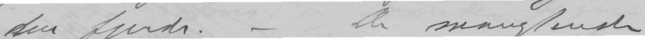den fjerde. - De manglende
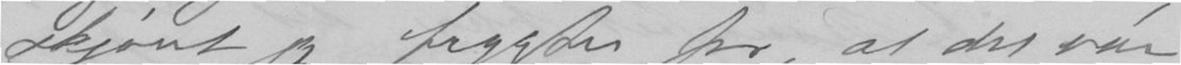skjønt jeg frygter for, at det var
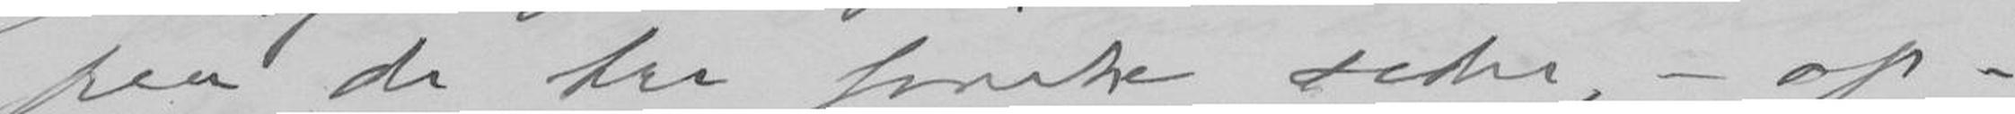paa de tre første sider, - op-
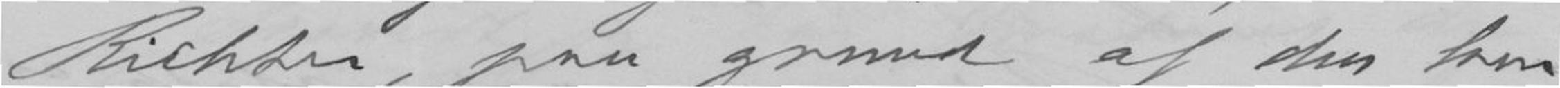Richter, paa grund af den lave
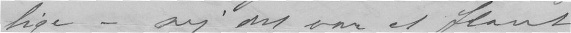lige - sig det var et flaut
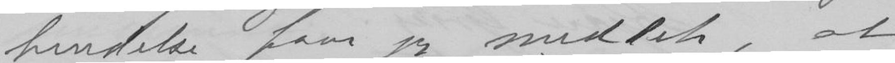sendelse faar jeg med det, at
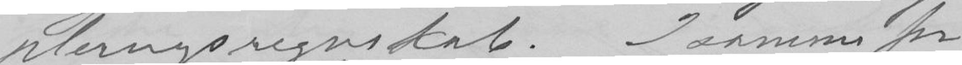leringsregnskab. I samme for-
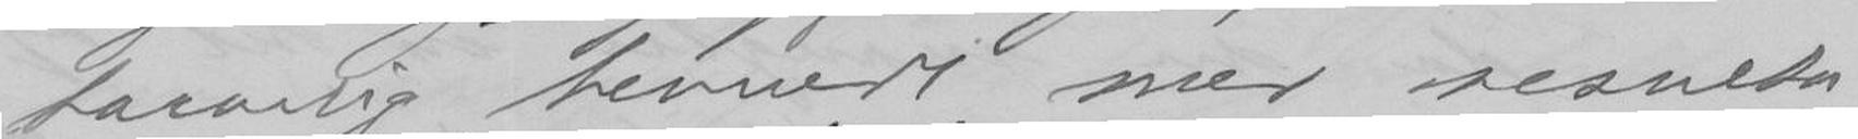tarvelig hernede med resulta-
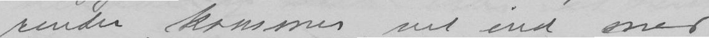renter, kommer vel ind med
